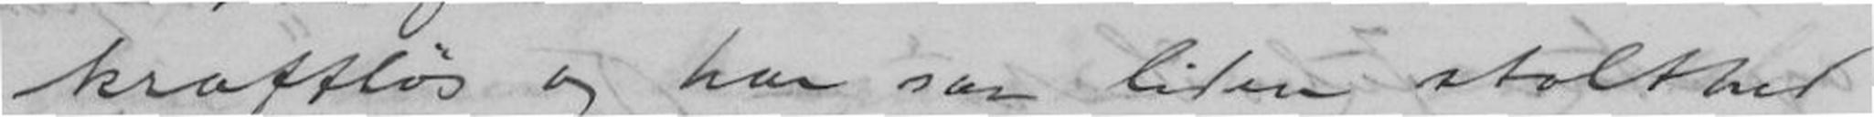kraftløs og har saa liden stolthed
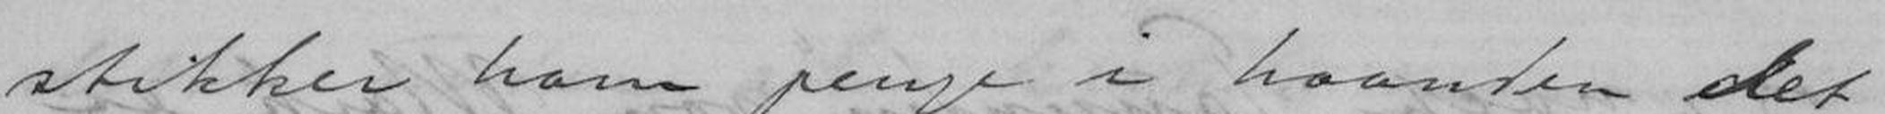stikker ham penge i haanden det
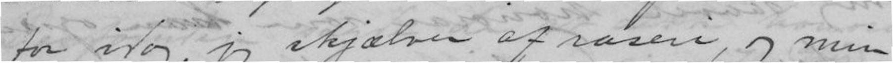for idag, jeg skjælver af raseri, og min
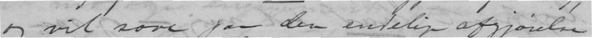og vil sove paa den endelige afgjørelse
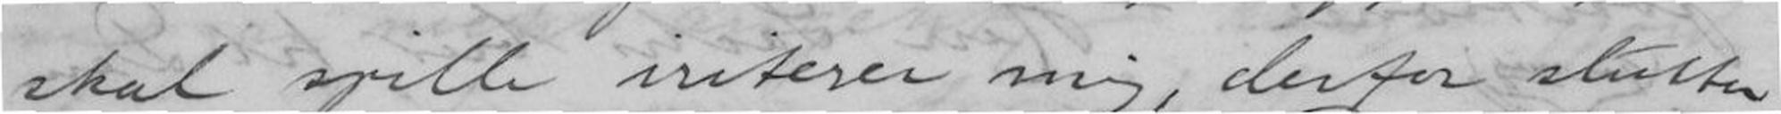skal spille iriterer mig, derfor slutter
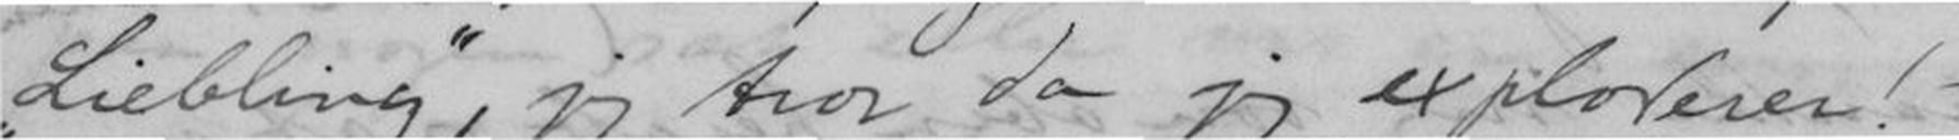"Liebling", jeg tror da jeg exploderer! -
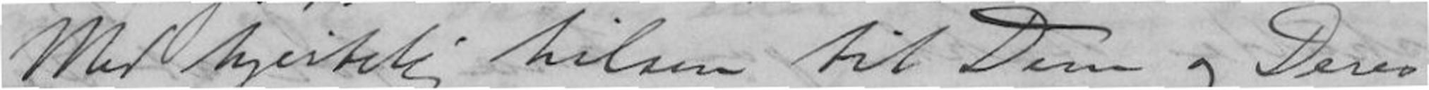Med hjertelig hilsen til Dem og Deres
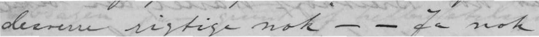desverre rigtige nok. - - Ja nok
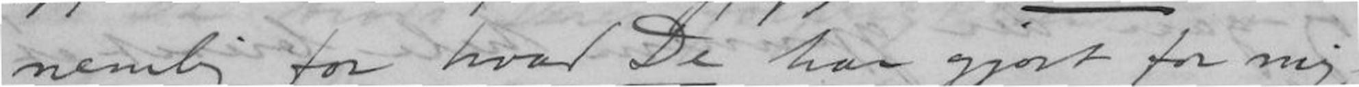nemlig for hvad De har gjort for mig, -
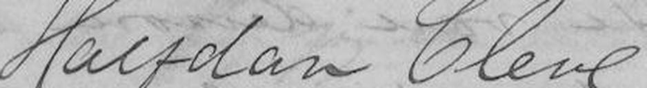Halfdan Cleve.
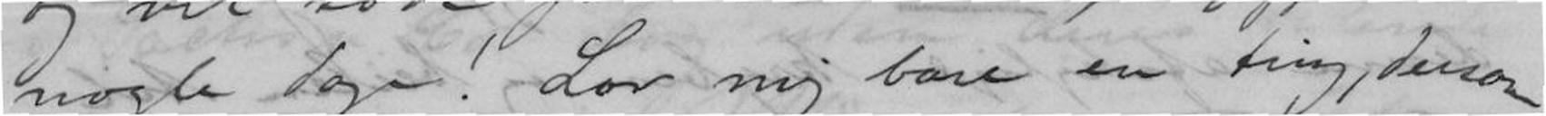nogle dage! Lov mig bare en ting, dersom
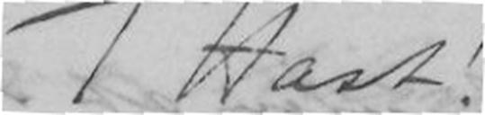I Hast!
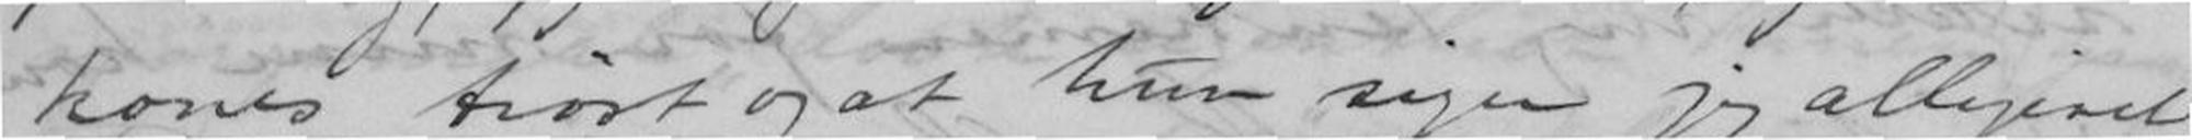kones trøst og at hun siger jeg alligevel
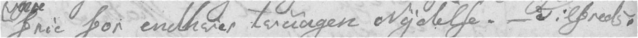være frie for endhver tvungen Nydelse. – Tilfreds.
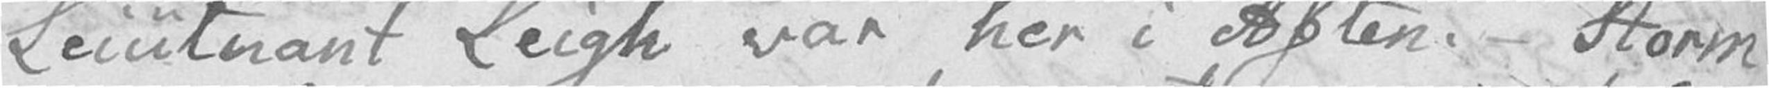Leiutnant Leigh var her i Aften. – Storm
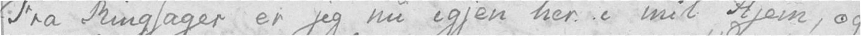Fra Ringsager er jeg nu igjen her i mit Hjem, og
